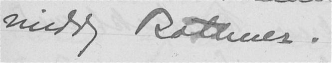middag Bothner.
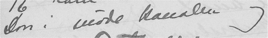Don i møde kanalen og
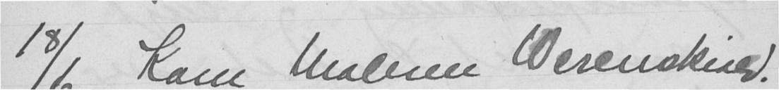18/6 Kom Maleren Werenskiold.
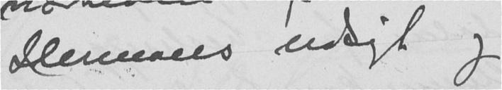Hermans udsigt og
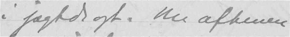i jagtdragt. Om aftenen
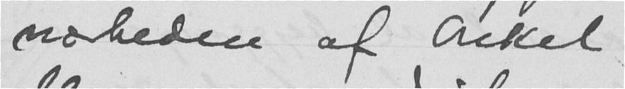nærheden af Onkel
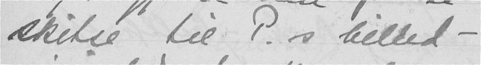skitse til P.s billed -
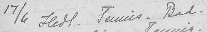17/6 Hedt. Tennis. Bad.
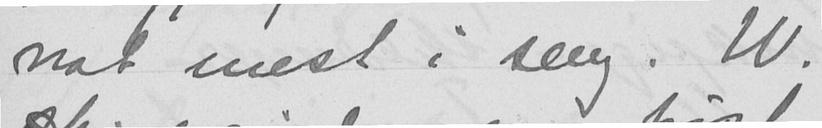nat mest i seng. W.
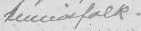tennisfolk.
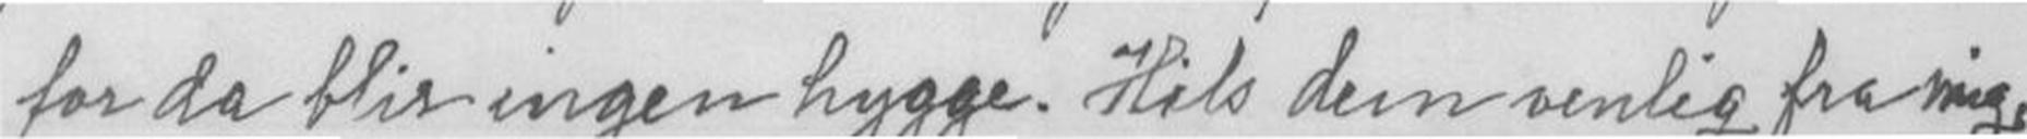for da blir ingen hygge. Hils dem venlig fra mig,
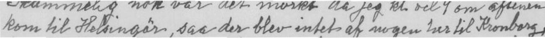kom til Helsingør, saa der blev intet af nogen her til Kronborg,
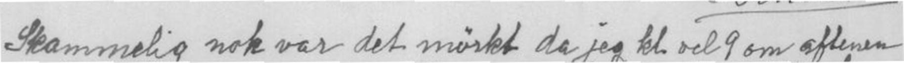Skammelig nok var det mørkt da jeg kl vel 9 om aftenen
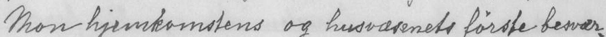Mon hjemkomstens og husvæsenets første besvær-
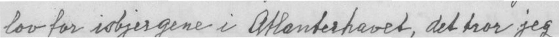lov for isbjergene i Atlanterhavet, det tror jeg
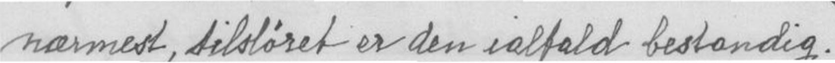nærmeste, tilsløret er den ialfald bestandig.
